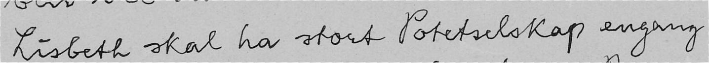Lisbeth skal ha stort Potetselskap engang
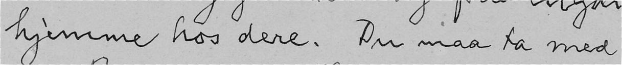hjemme hos dere. Du maa ta med
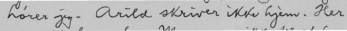hører jeg. Arild skriver ikke hjem. Her
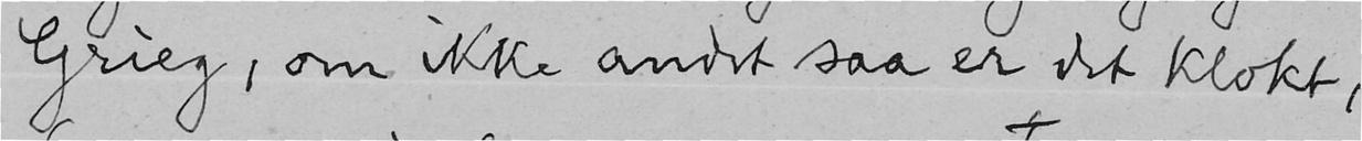Grieg, om ikke andet saa er det klokt,
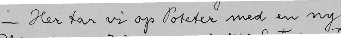- Her tar vi op Poteter med en ny
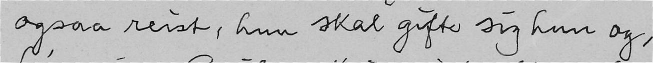ogsaa reist, hun skal gifte sig hun og,
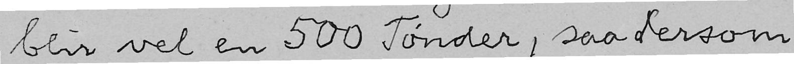blir vel en 500 Tønder, saa dersom
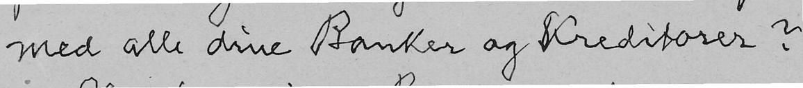med alle dine Banker og Kreditorer?
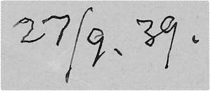27/9. 39.
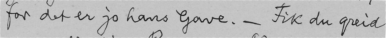for det er jo hans Gave. - Fik du greiet
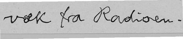væk fra Radioen.
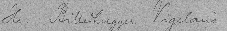Hr. Billedhugger Vigeland
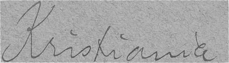Kristiania
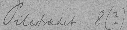Pilestrædet 8 (?)
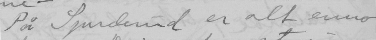På Sjurderud er alt enno
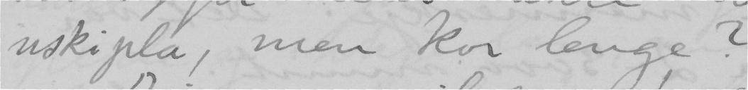uskipla, men kor lenge?
